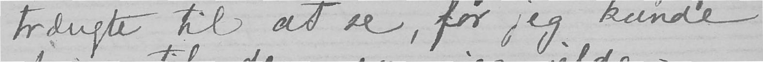trængte til at se, før jeg kunde
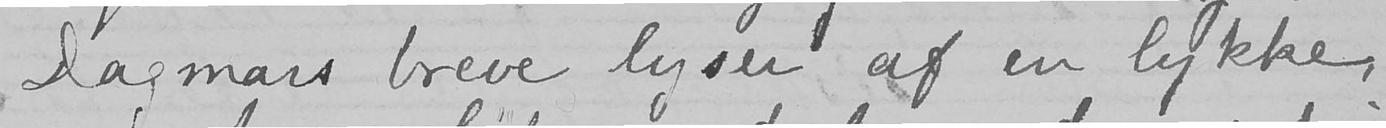Dagmars breve lyser af en lykke,
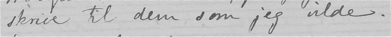skrive til dem som jeg vilde.
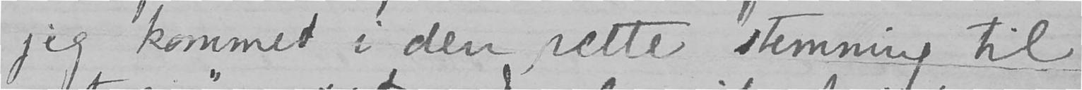jeg kommet i den rette stemning til
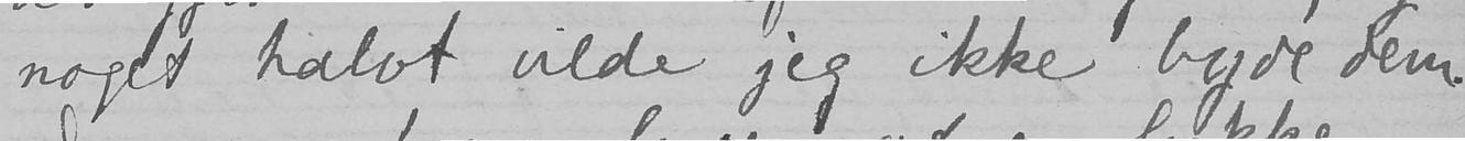noget halvt vilde jeg ikke byde dem.
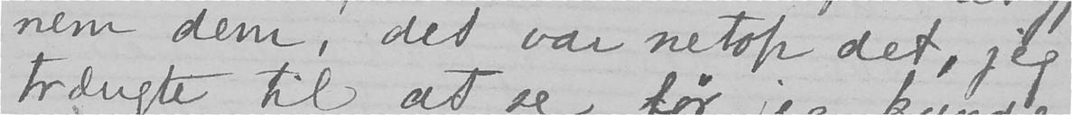nem dem, det var netop det, jeg
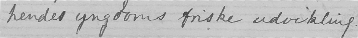hendes yngdoms friske udvikling.
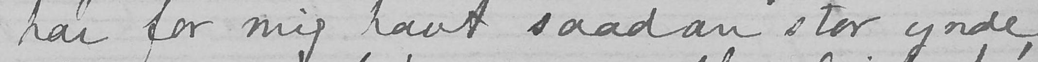har for mig havt saadan stor ynde,
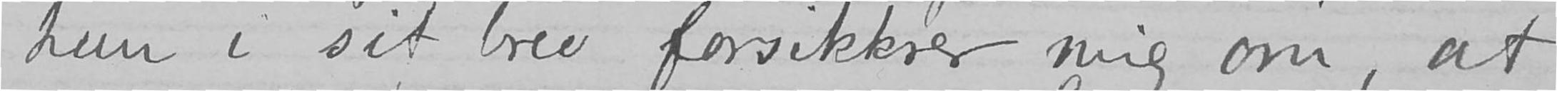hun i sit brev forsikkrer mig om, at
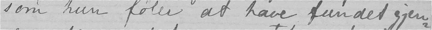som hun føler at have fundet gjen-
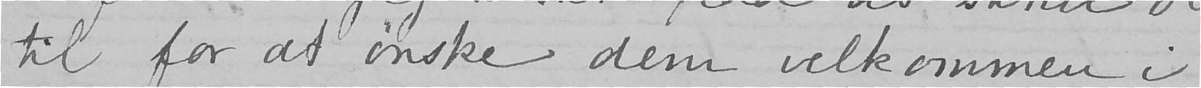til for at ønske dem velkommen i
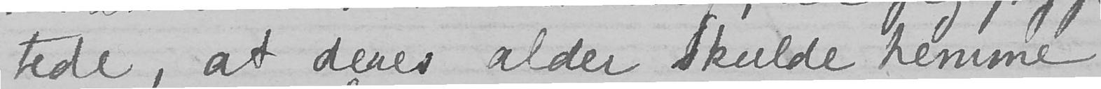tede, at deres alder skulde hemme
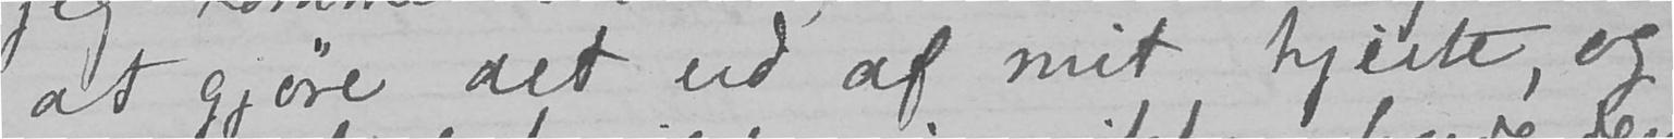at gjøre det ud af mit hjerte, og
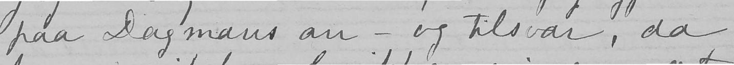paa Dagmars an - og tilsvar, da
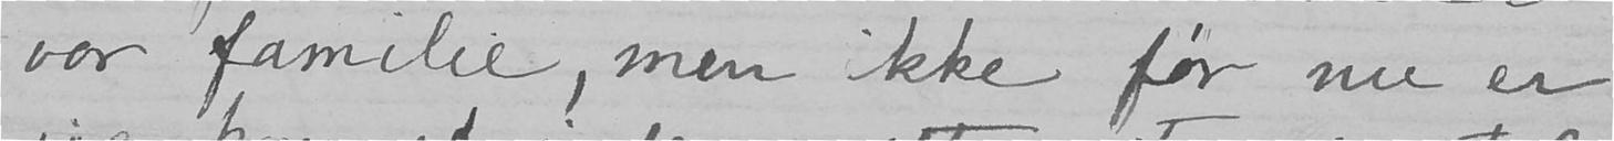vor familie, men ikke før nu er
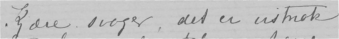Kjære svoger, det er vistnok
